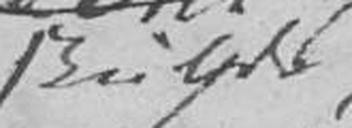skulde
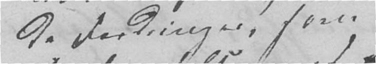de Fordringer, som
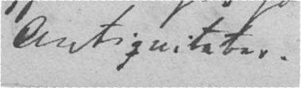Antiqviteter.
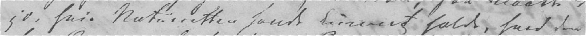jo, hvis Naturretten havde kunnet holde, hvad den
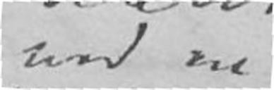ved en
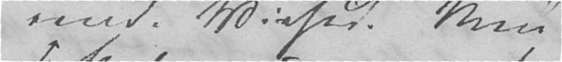rende Vished. Men
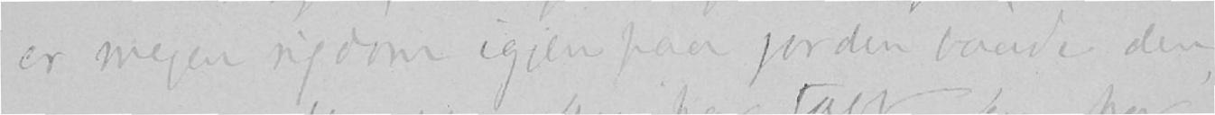er meget rigdom igjen paa jorden baade den
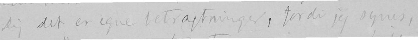Dig det er egne betragtninger, fordi jeg synes,
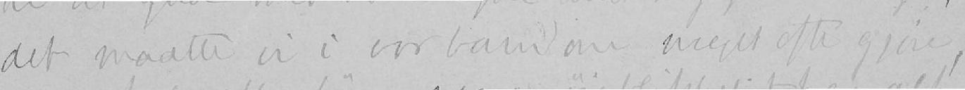det maatte vi i vor barndom meget ofte gjøre,
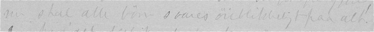nu skal alle børn svares øieblikkelig paa alt.
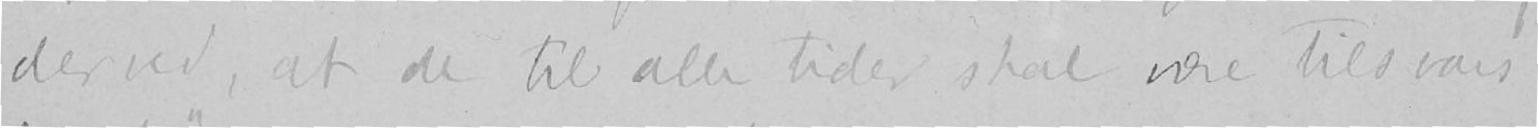derved, at de til alle tider skal være tilsvars
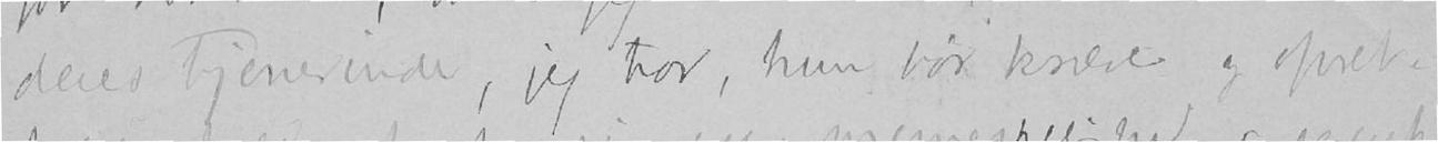deres tjenerinde, jeg tror, hun bør kreve og opret-
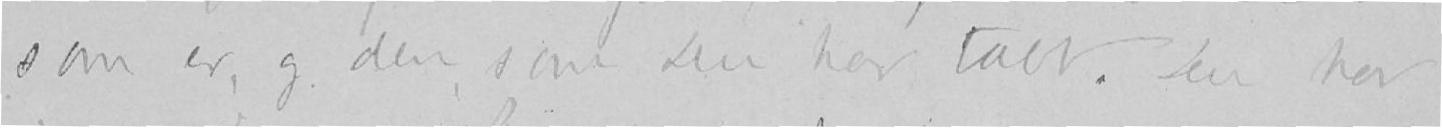som er, og den som Du ikke har tabt. Du har
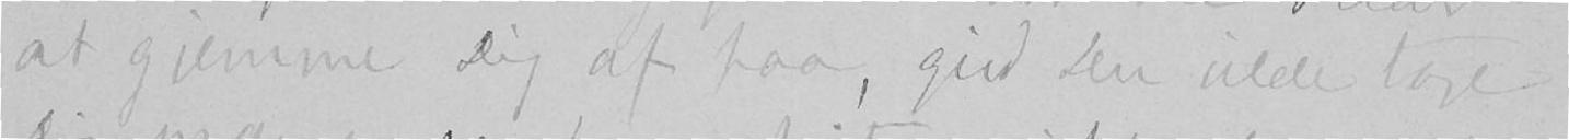at gjemme Dig af paa, gid Du vilde tage
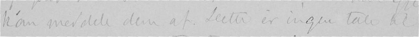kan meddele dem af. Dette er ingen tale til
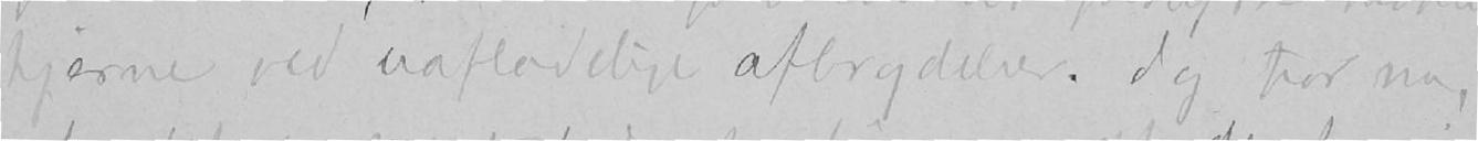hjerne ved uafladelige afbrydelser. Jeg tror nu,
